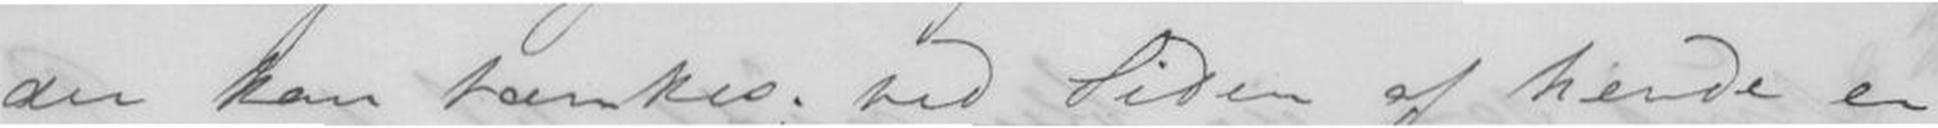der kan tænkes; ved Siden af hende er
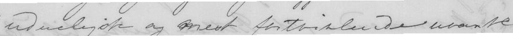udueligste og mest fortvivlende uvante,
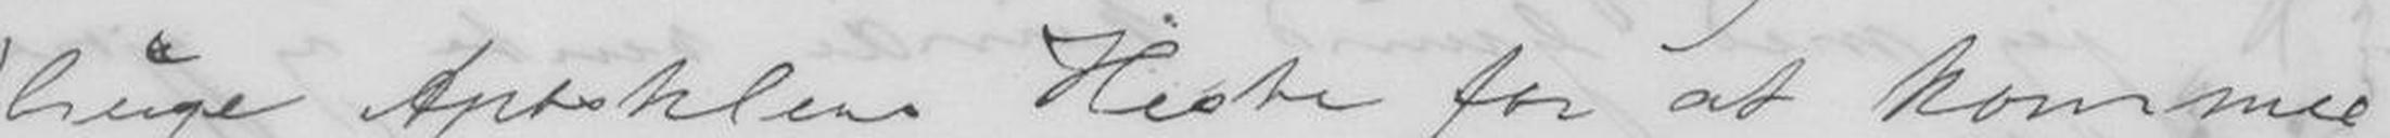bruge Apostelens Heste for at komme
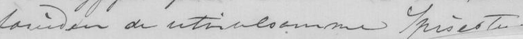foruden de utvivlsomme Spisest
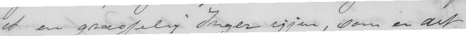et en græsselig Inger igjen, som er det
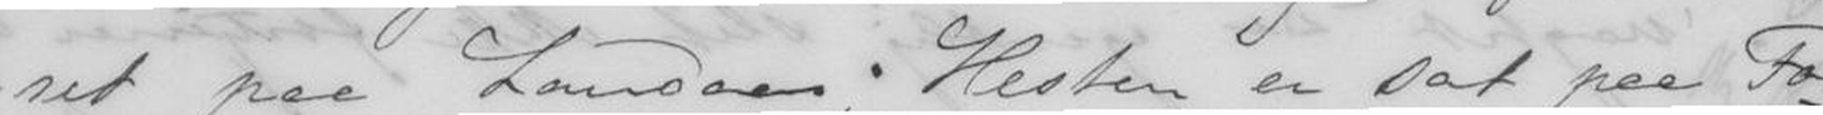ret paa Landaas; Hesten er sat paa Fo-
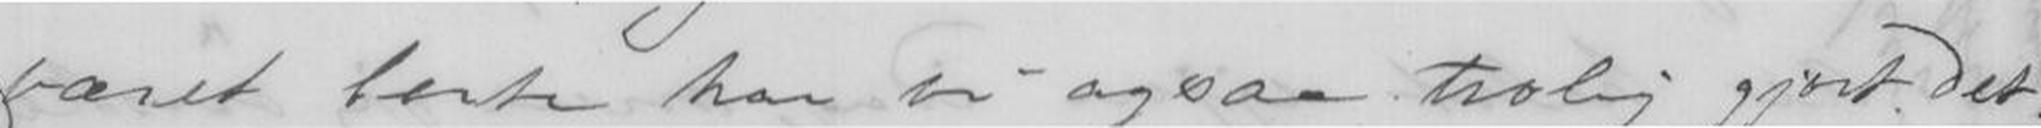været borte har vi ogsaa trolig gjort det;
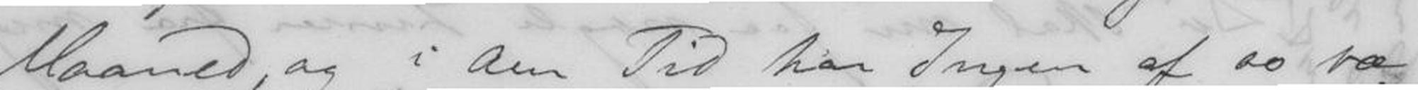Maaned, og i den Tid har Ingen af os væ-
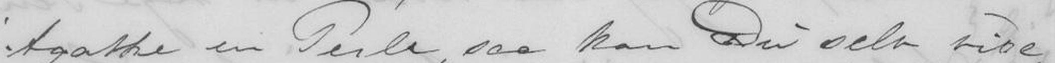Agathe en Perle, saa kan Du selv vide;
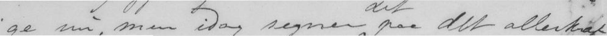ge nu, men idag regner paa det allerkraf-
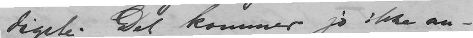digste. Det kommer jo ikke an-
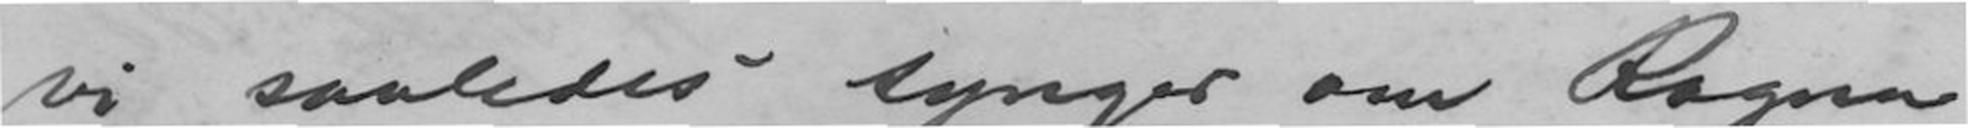vi saaledes synger om Ragna
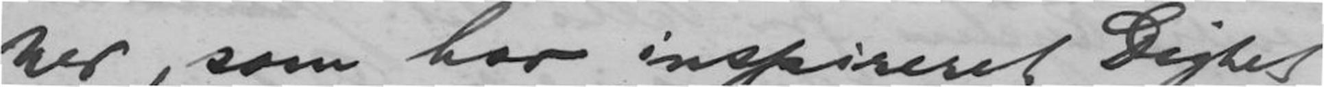ner, som har inspireret Digtet
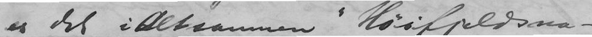er det i Altsammen "Høifjeldsna-
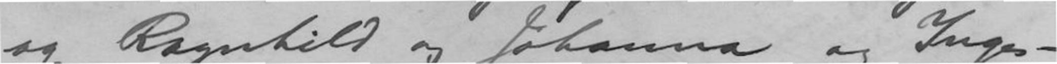og Ragnhild og Johanne og Inge-
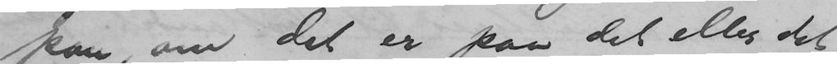paa, om det er paa det eller det
